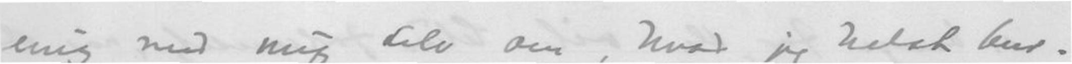enig med mig selv om, hvad jeg helst bur-
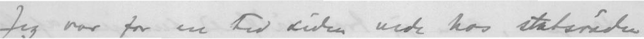Jeg var for en tid siden nede hos statsråden
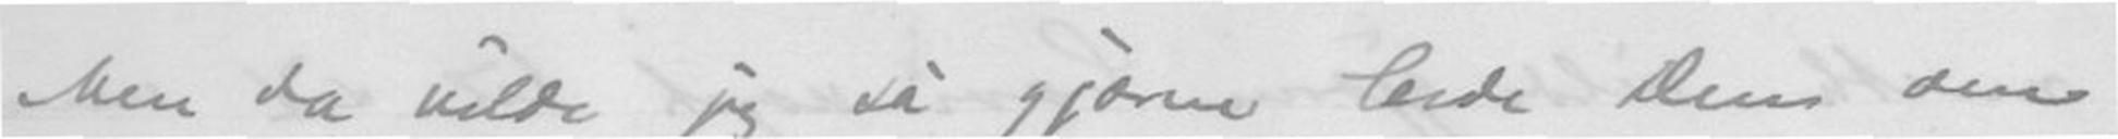Men da vilde jeg så gjerne bede Dem om
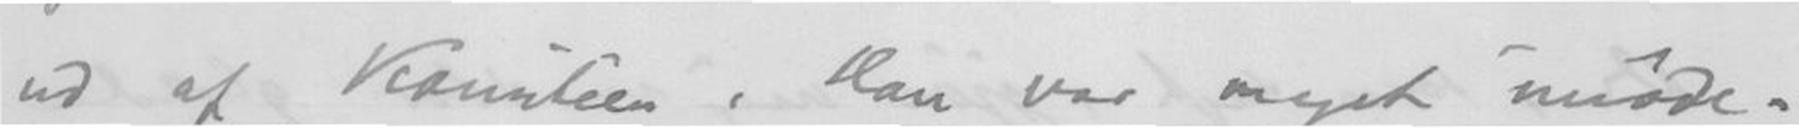ud af Komitéen. Han var meget imøde-
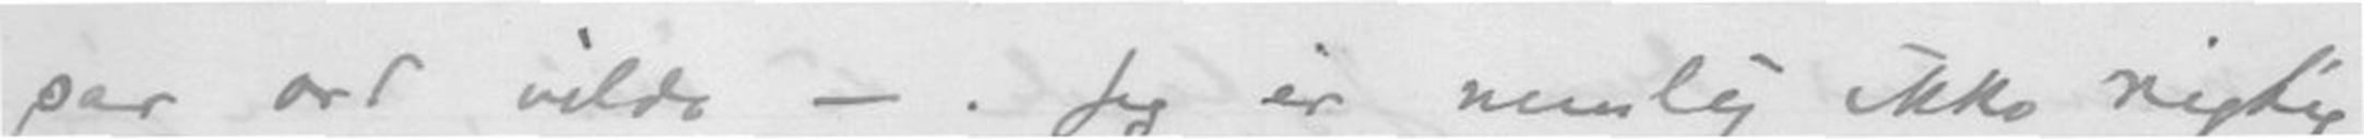par ord vilde -. Jeg er nemlig ikke rigtig
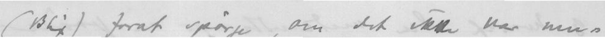(Blix) forat spørge, om det ikke var mu-
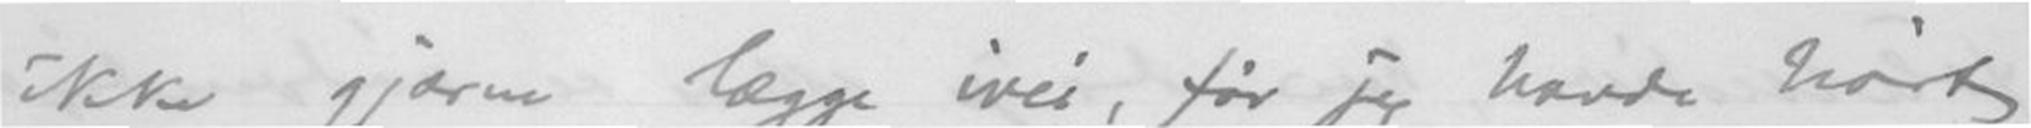ikke gjærne lægge ivei, før jeg havde hørt
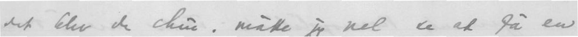det blev de chin. måtte jeg vel se at få en
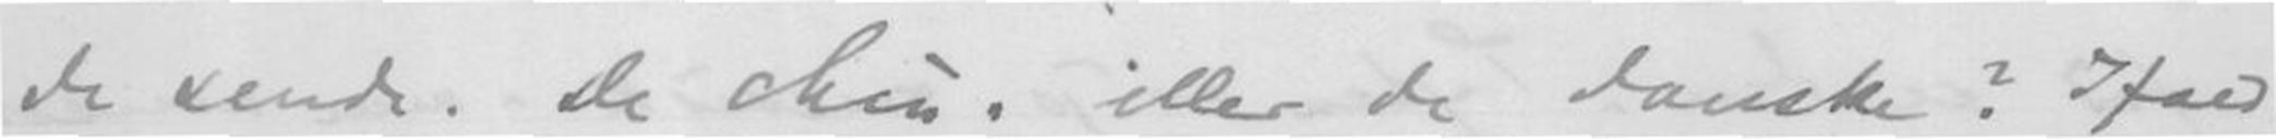de sende. De chin. eller de danske? Ifald
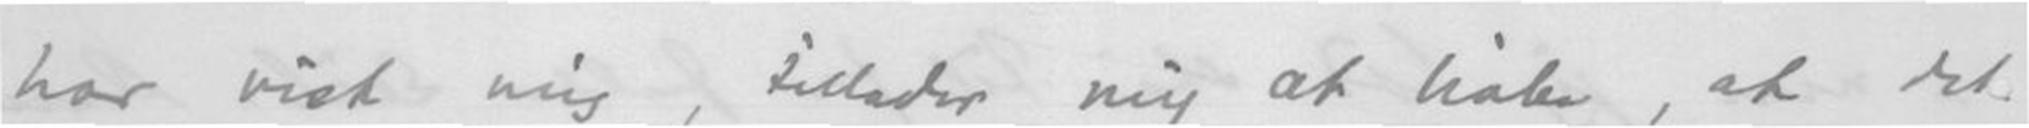har vist mig, tillader mig at håbe, at
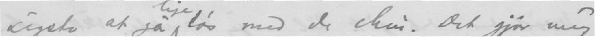sigste at gå lige løs med de Chin. Det gjør mig
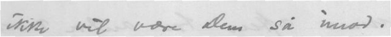det ikke vil være Dem så imod.
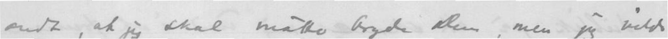ondt, at jeg skal måtte bryde Dem, men jeg vilde
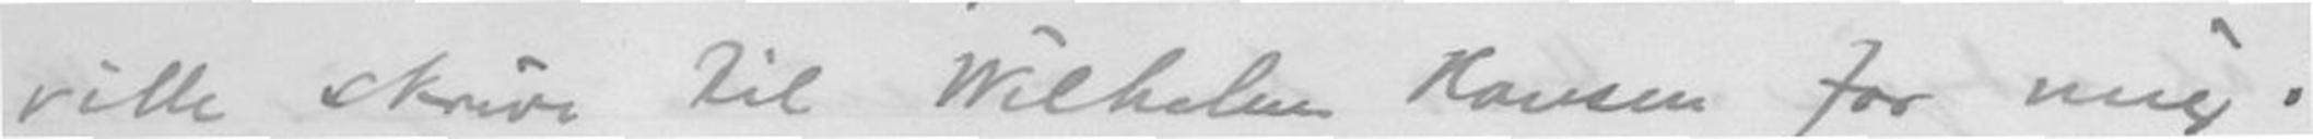ville skrive til Wilhelm Hansen for mig!
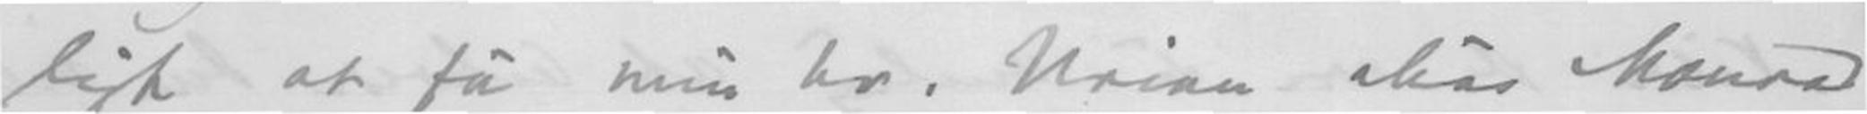ligt at få min hr. Novian alias Monrad
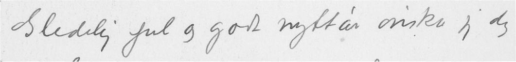Gledelig jul og godt nyttår ønsker jeg dig
